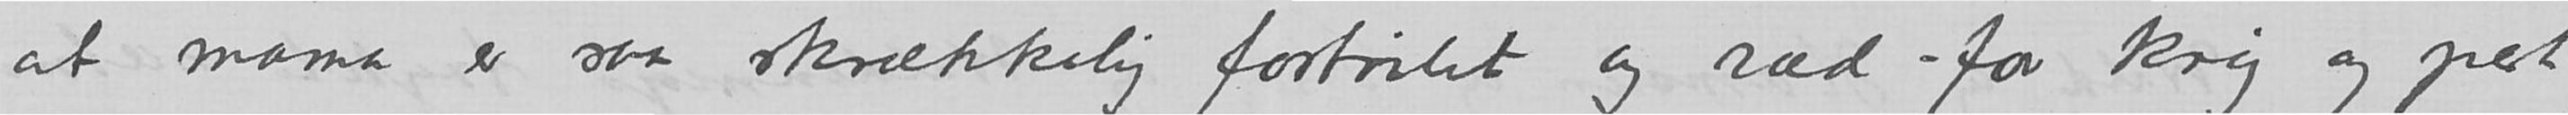at mama er saa skrækkelig fortvilet og ræd - for krig og pest
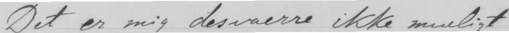Det er mig desværre ikke muligt
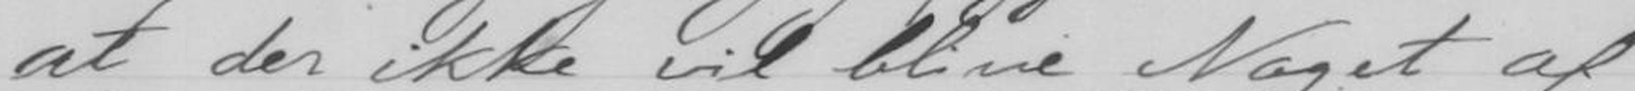at der ikke vil blive Noget af
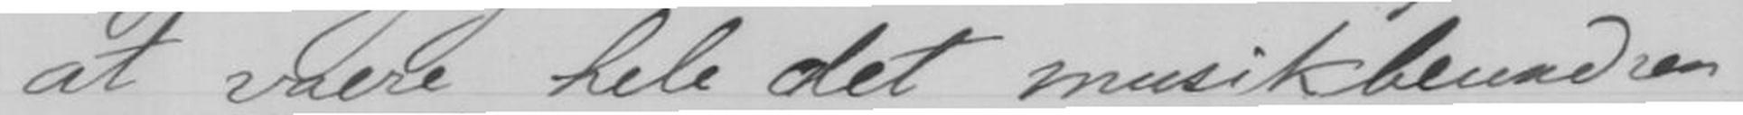at vaere hele det musikbeundren
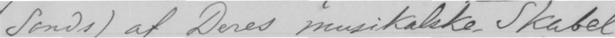Sonds) af Deres musikalske Skabel-
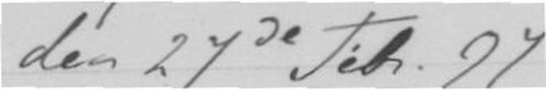den 24 Feb. 97
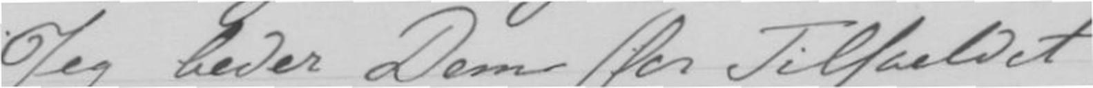Jeg beder Dem (for Tilfaeldet
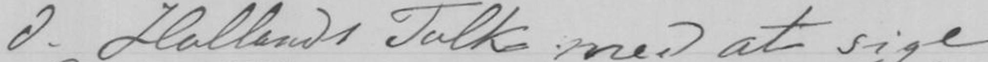d. Hollands Tolk med at sige
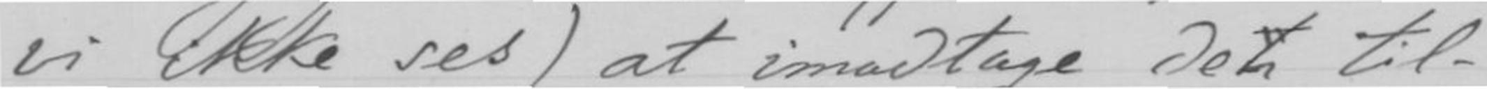vi ikke ses) at imodtage Den til-
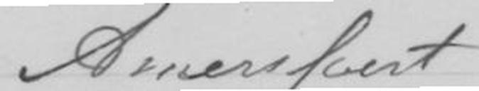Amersfaest
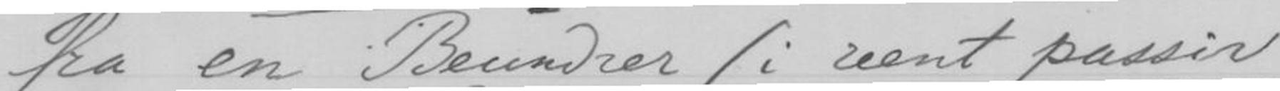fra en Beundrer (i reent passiv
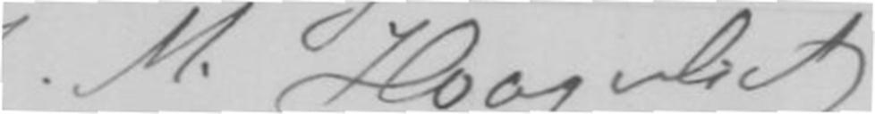J.M.Hoogenlict
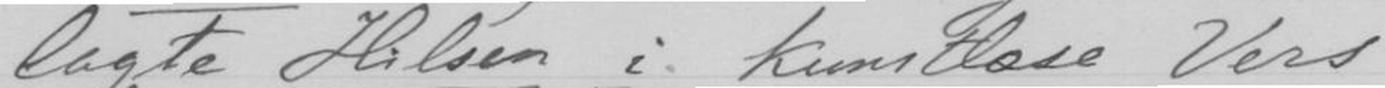lagte Hilsen i kunstløse Vers
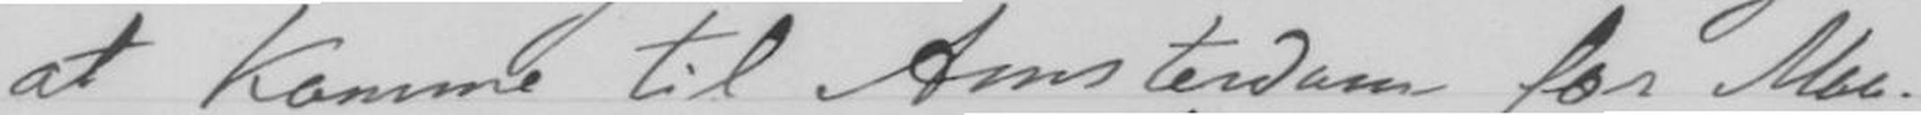at komme til Amsterdam før Maa-
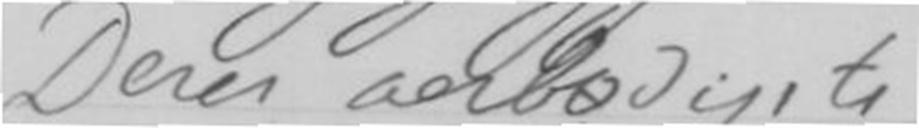Deres ærbødigste
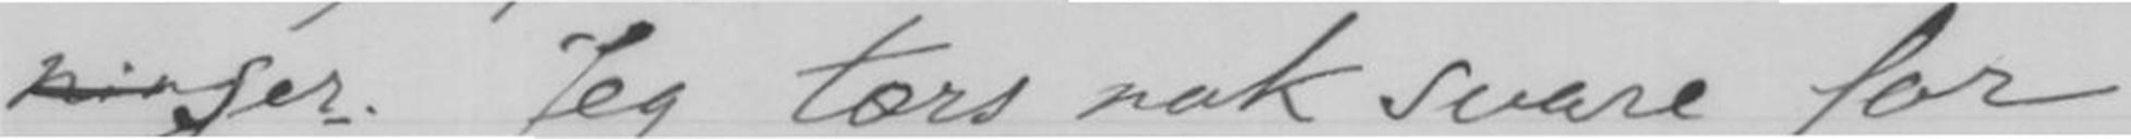ser. Jeg tørs nok svare for
